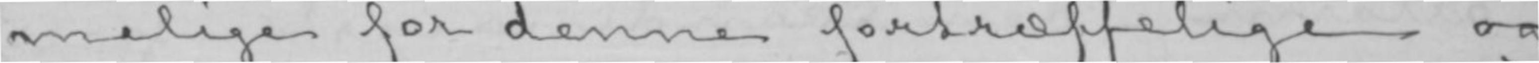melig for denne fortræffelige og
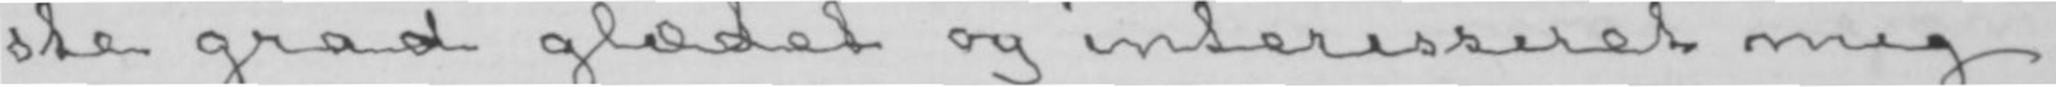ste grad glædet og interesseret mig.
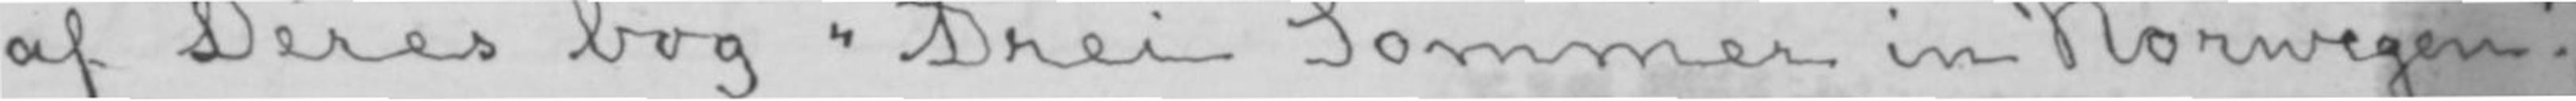af Deres bog «Drei Sommer in Norwegen».
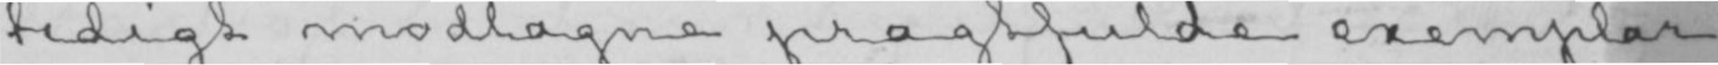tidigt modtagne pragtfulde exemplar
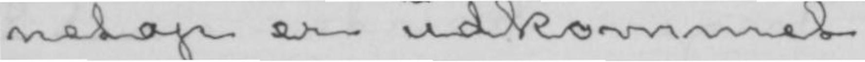netop er udkommet.
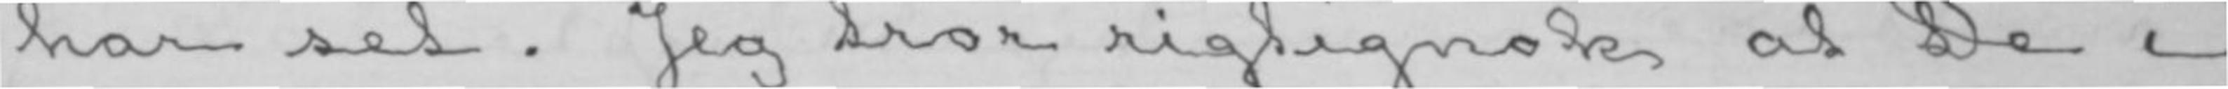har set. Jeg tror rigtignok at De i
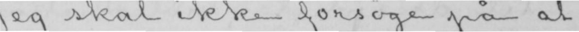Jeg skal ikke forsøge på at
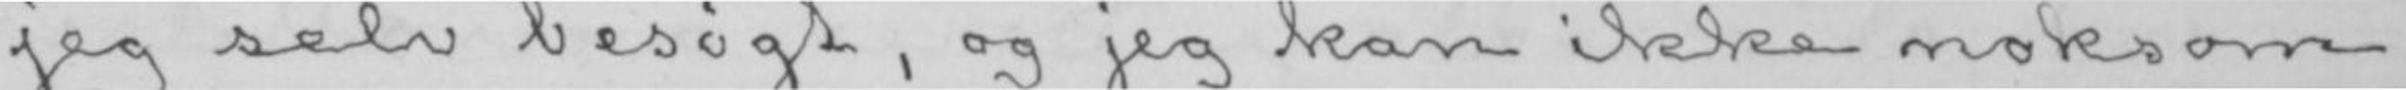jeg selv besøgt, og jeg kan ikke noksom
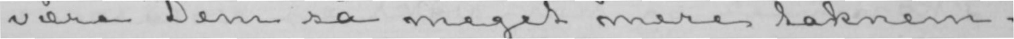være Dem så meget mere taknem-
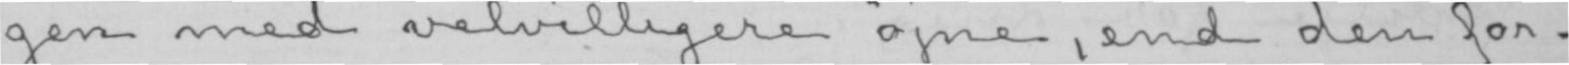gen med velvilligere øjne, end den for-
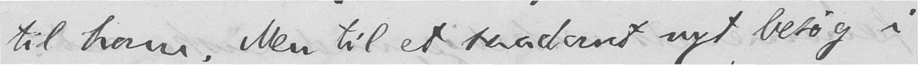til ham. Men til et saadant nyt besøg i
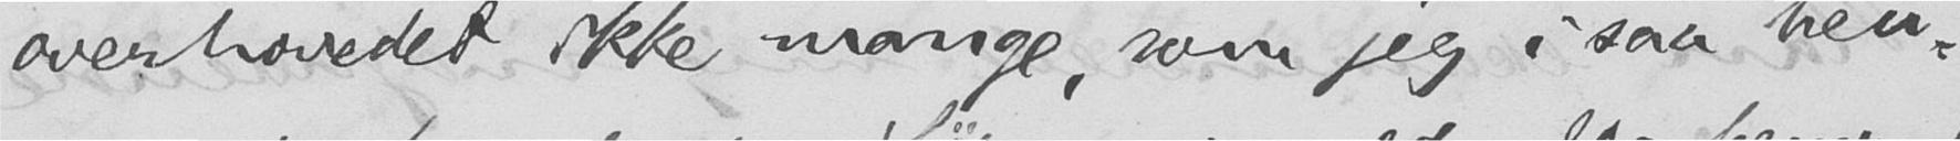overhovedet ikke mange, som jeg i saa hen-
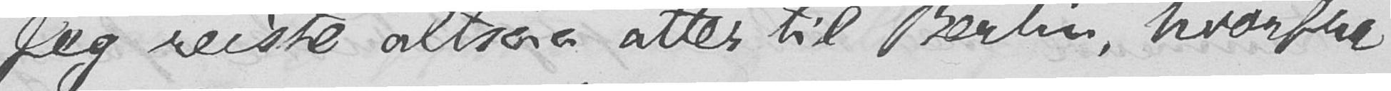Jeg reiste altsaa atter til Berlin, hvorfra
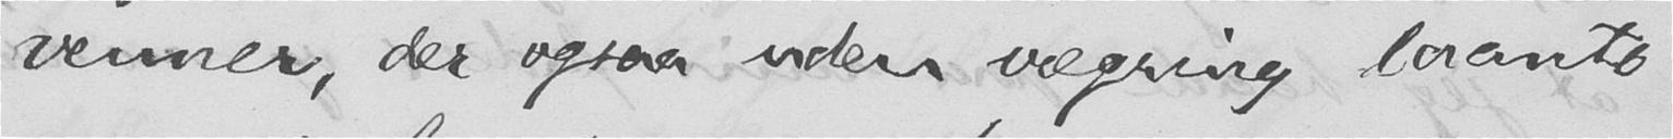venner, der ogsaa uden vægring laante
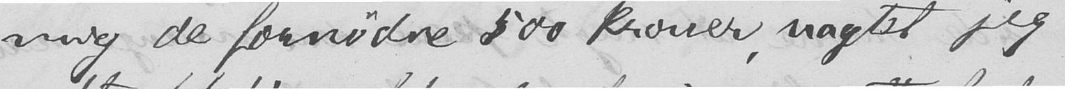mig de fornødne 500 kroner, uagtet jeg
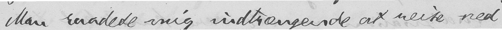Man raadede mig indtrængende at reise ned
